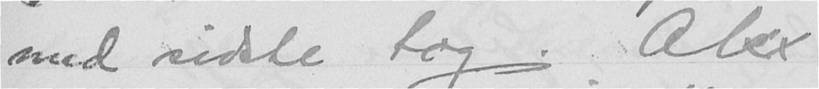med sidste tog. Alex
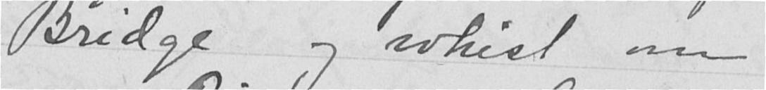Bridge og whist om
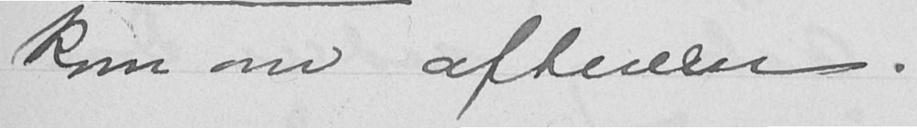kom om aftenen.
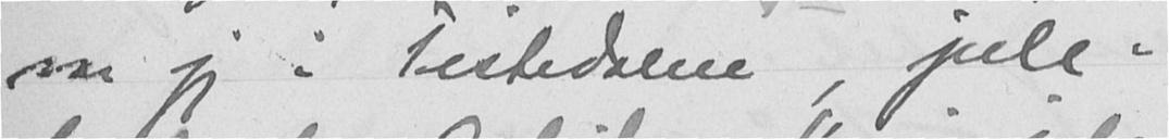var jeg i Tistedalen, jule-
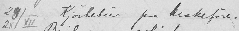28/XII Kjørtetur paa krakeføre.
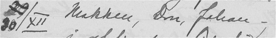30/XII Makken, Don, Johan og
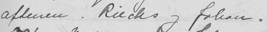aftenen. Riecks og Johan.
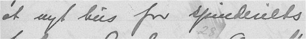et nyt hus for spinderiets
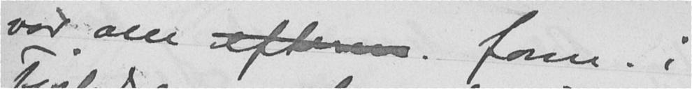var om efterm. form. i
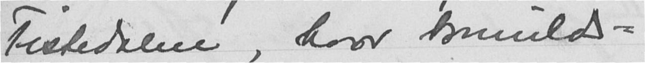Tistedalen, hvor bomulds-
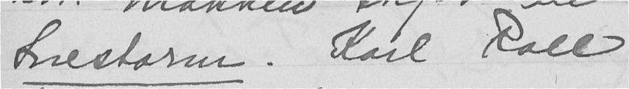Snestorm. Karl Roll
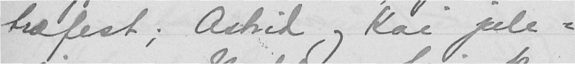træfest; Astrid og Kai jule-
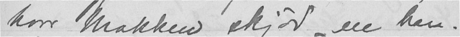hvor Makken skjød en hare.
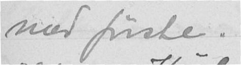med første.
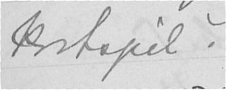kortspil.
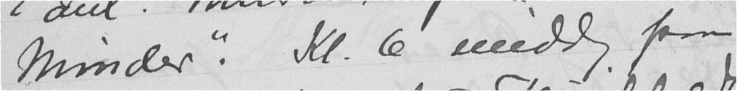Minder". Kl. 6 middag paa
